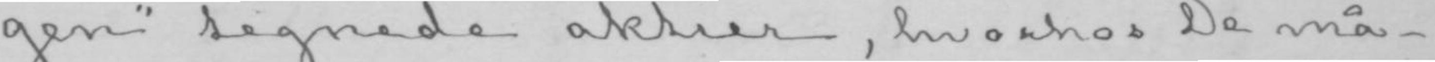gen» tegnede aktier, hvorhos De må-
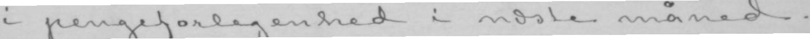i pengeforlegenhed i næste måned.
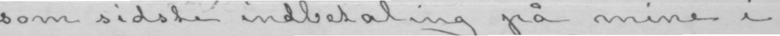som sidste indbetaling på mine i
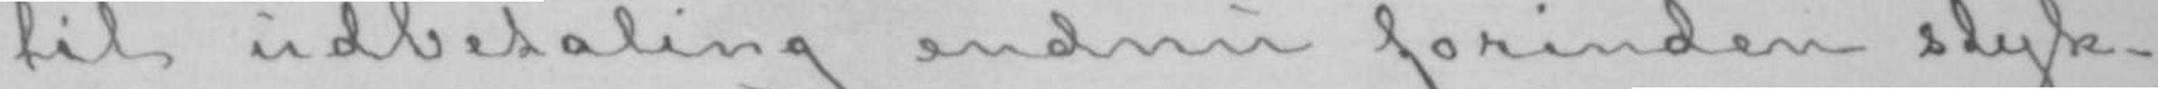til udbetaling endnu forinden styk-
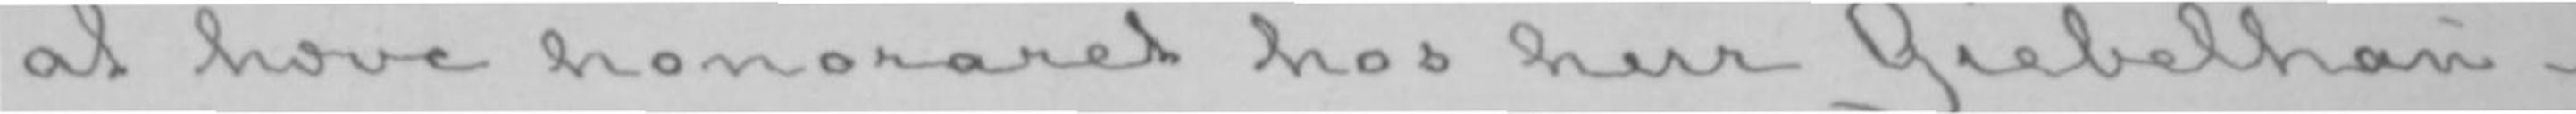at hæve honoraret hos herr Giebelhau-
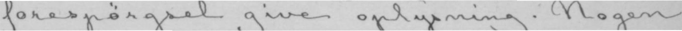forespørgsel give oplysning. Nogen
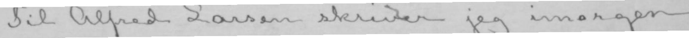Til Alfred Larsen skriver jeg imorgen
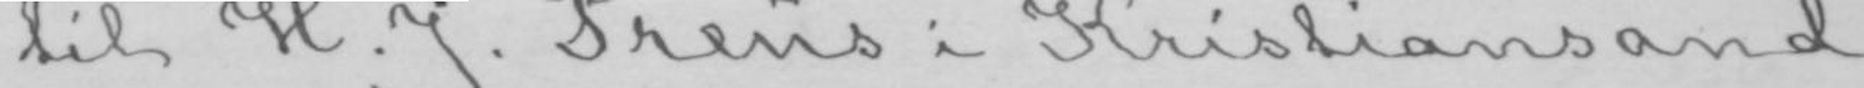til H. J. Preus i Kristiansand
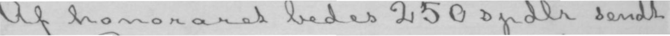Af honoraret bedes 250 spdlr sendt
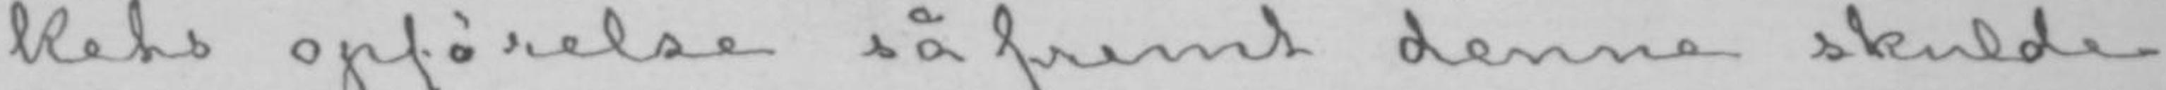kets opførelse såfremt denne skulde
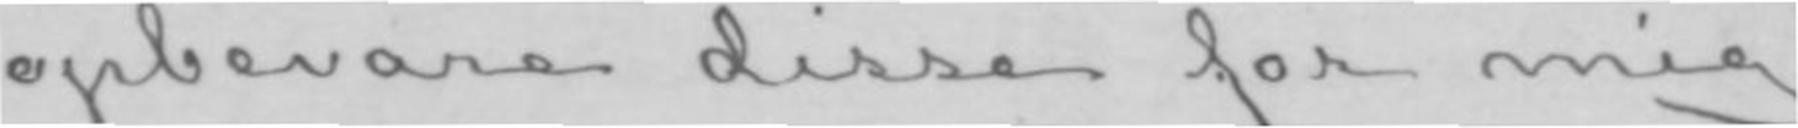opbevare disse for mig.
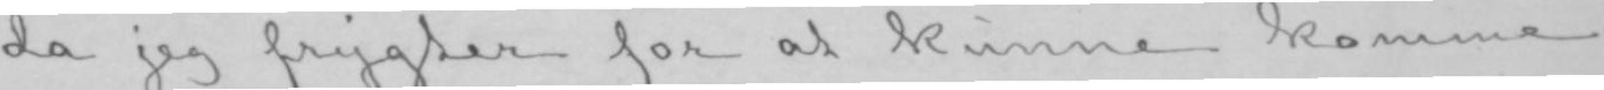da jeg frygter for at kunne komme
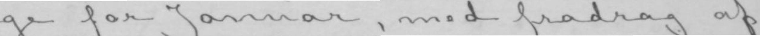gage for Januar, med fradrag af
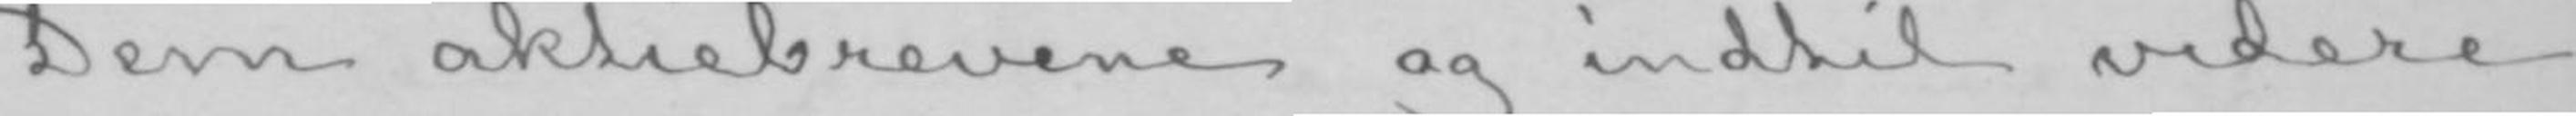Dem aktiebrevene og indtil videre
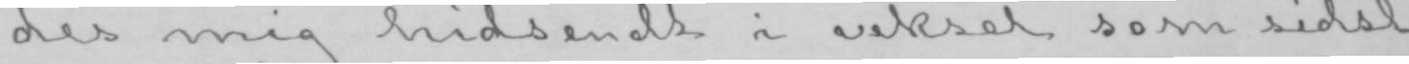des mig hidsendt i veksel som sidst,
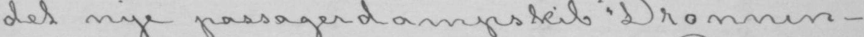det nye passagerdampskib «Dronnin-
